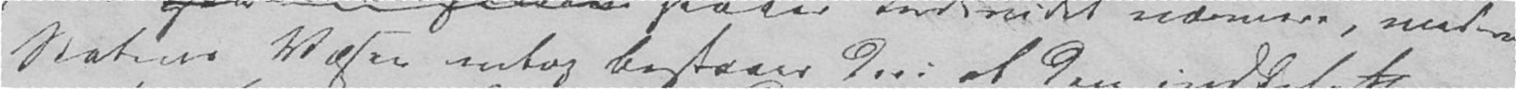Statens Væsen endog bestaaer deri at den indbefatter og
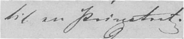til en Privatret.
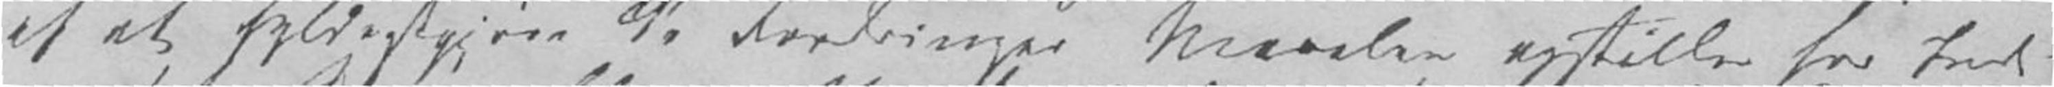af at fyldestgjøre de Fordringer Moralen opstiller for Indi-
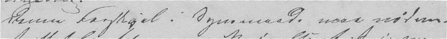Denne Forskjel i Synsmaade maa nødven-
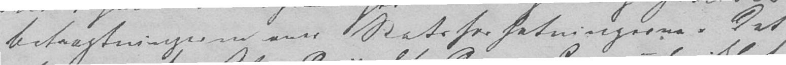Betragtningerne om Statsforfatningerne. Det
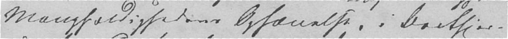Mangfoldighedens Ophævelse, i Bortfjer-
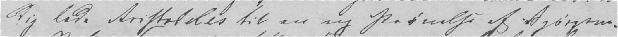dig lede Aristoteles til en ny Prøvelse af Spørgsm.
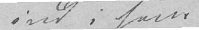ind i hans
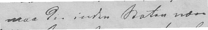maa der inden Staten være
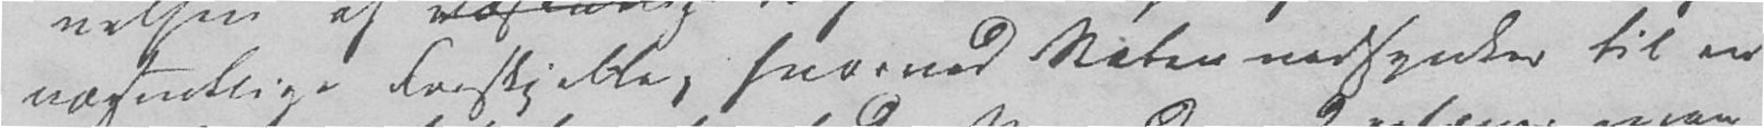væsentlige Forskjelle, hvorved Staten nedsynker til en
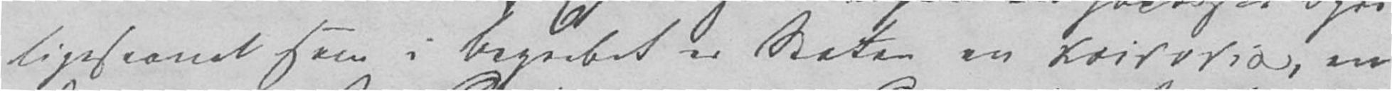ligesaavel som i Begrebet er Staten en gresk, en
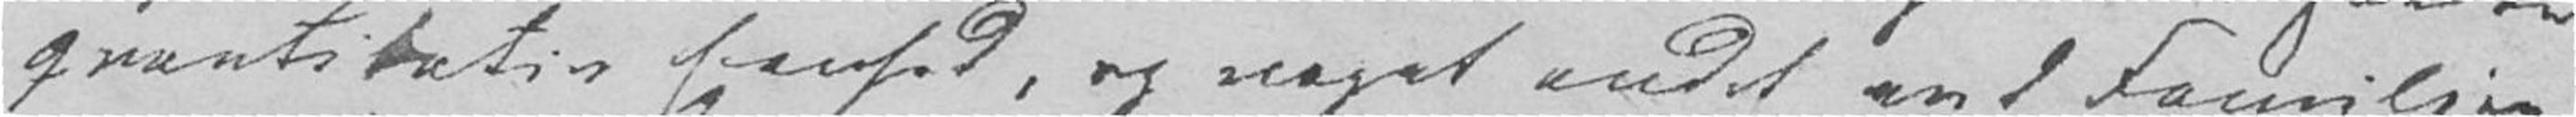qvantitativ Eenhed, og noget andet end Familie,
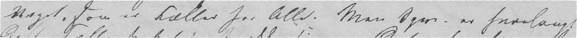Noget, som er Fælles for Alle. Men Spm. er hvorlangt
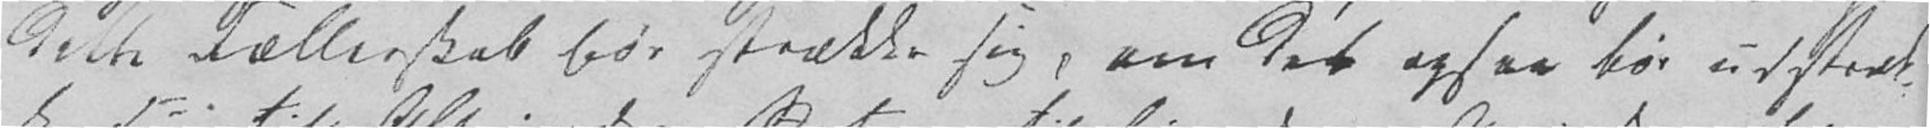dette Fællesskab bør strække sig, om det ogsaa bør udstræk-
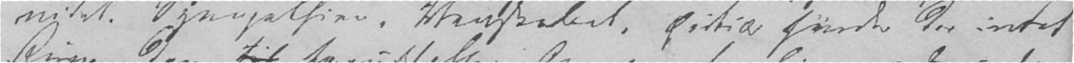videt. Sympathien, Venskabet, gresk finder der intet
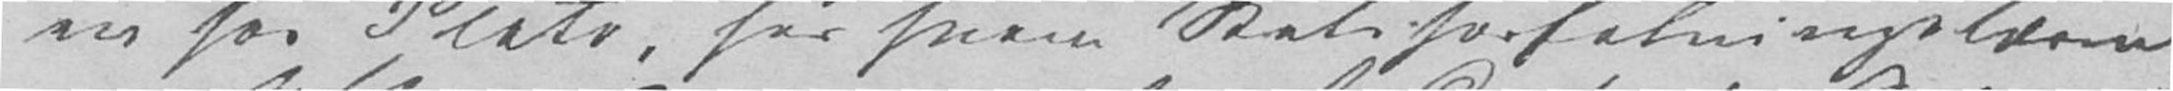en hos Plato, for hvem Statsforfatningslæren
